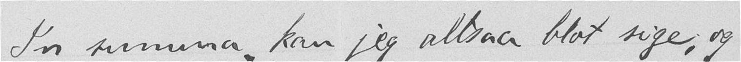In summa kan jeg altsaa blot sige, og
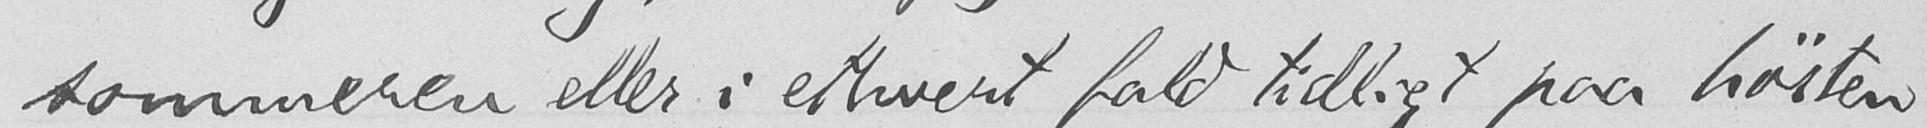sommeren eller i ethvert fald tidligt paa høsten
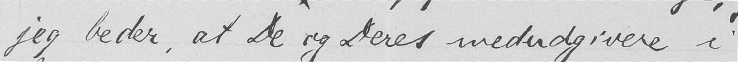jeg beder, at De og Deres medudgivere i
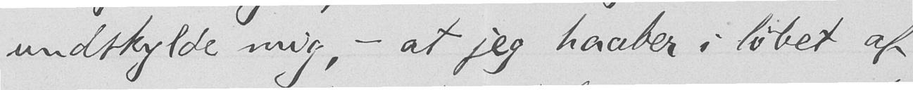undskylde mig, - at jeg haaber i løbet af
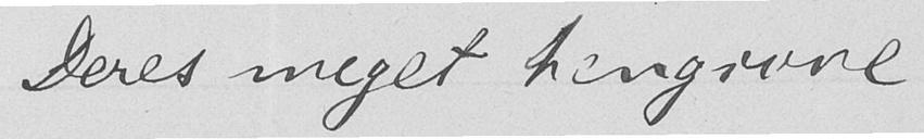Deres meget hengivne
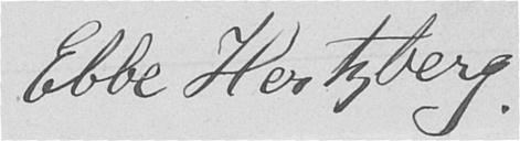Ebbe Hertzberg.
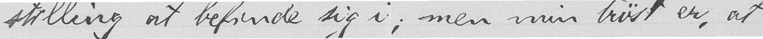stilling at befinde sig i; men min trøst er, at
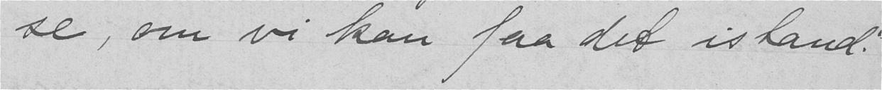se, om vi kan faa det istand."
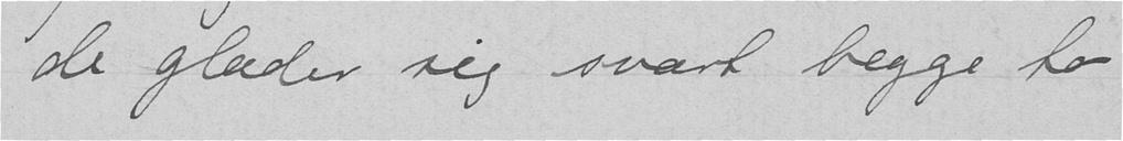de glæder sig svart begge to
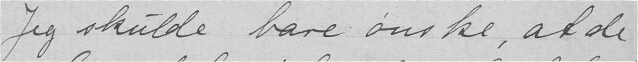Jeg skulde bare ønske, at de
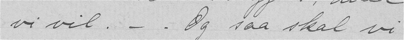vi vil. - Og saa skal vi
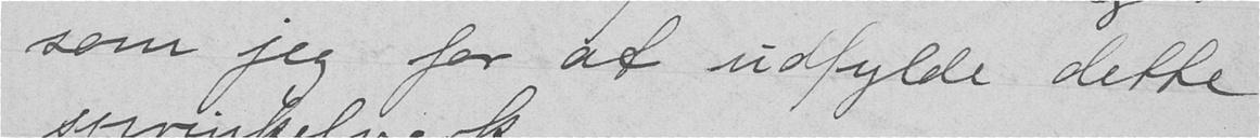som jeg for at udfylde dette
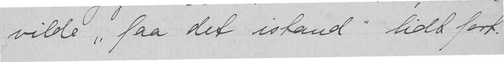vilde "faa det istand" lidt fort.
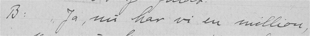B: "Ja, nu har vi en million,
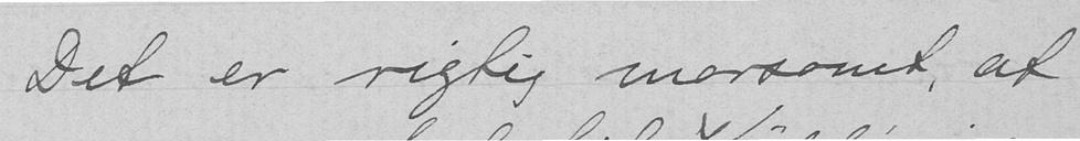Det er rigtig morsomt, at
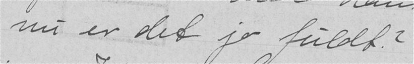nu er det jo fuldt?"
